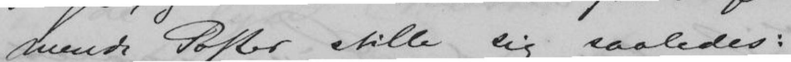mende Poster stille sig saaledes:
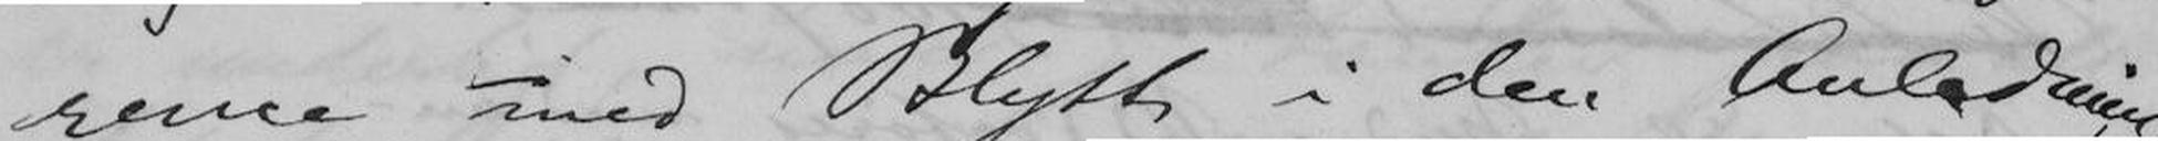rence med Blyth i den Andledning.
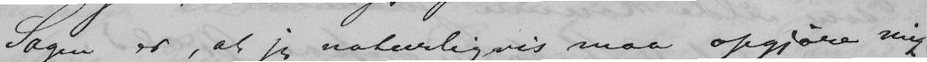Sagen er, at jeg naturligvis maa opgjøre mig
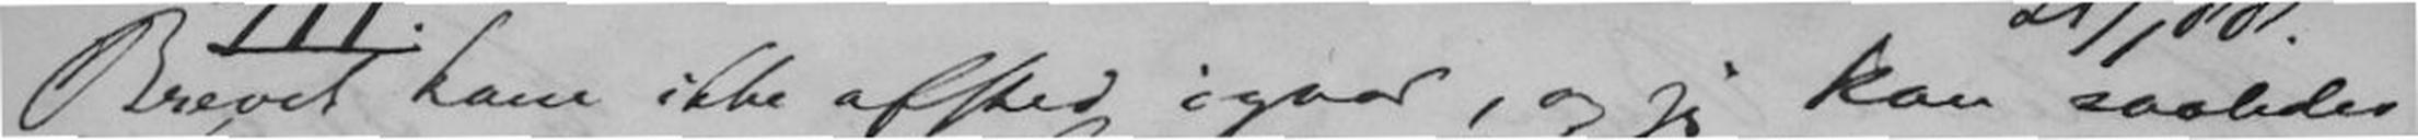Brevet kom ikke afsted igaar, og jeg kan saaledes
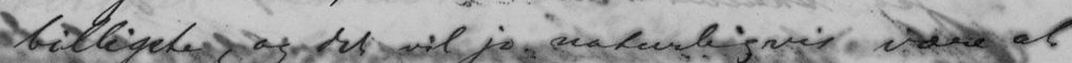billigste, og det vil jo naturligvis være at
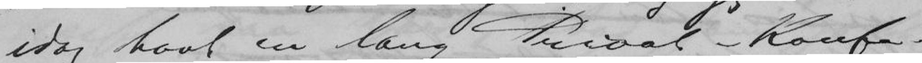idag havt en lang Privat-Konfe-
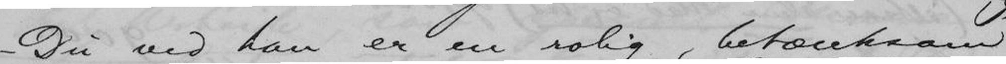-Du ved han er en rolig, betænksom
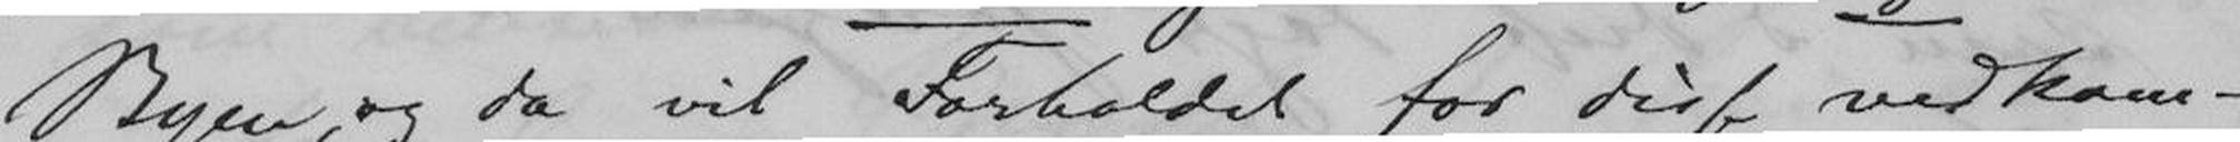Byen, og da vil Forholdet for disse vedkom-
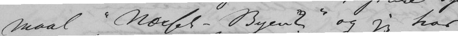maal "Næsset - Byen?" og jeg har
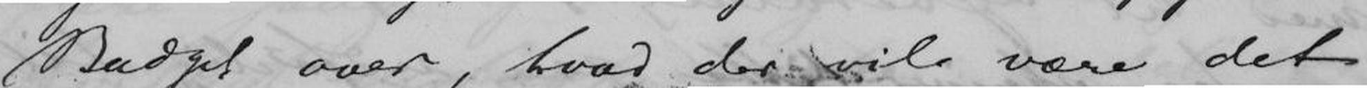Budget over, hvad der vil være det
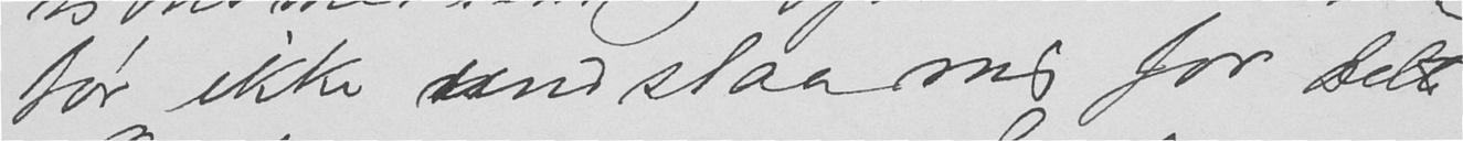tør ikke undslaa mig for dette
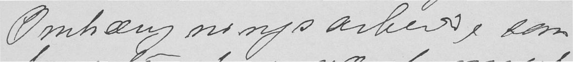Omhængningsarbeide som
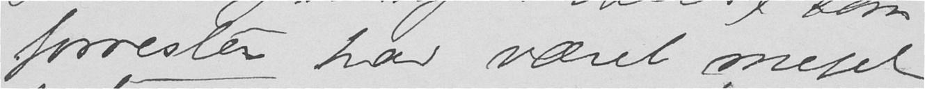forresten har været meget
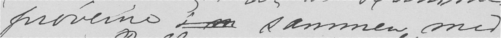prøverne i m sammen med
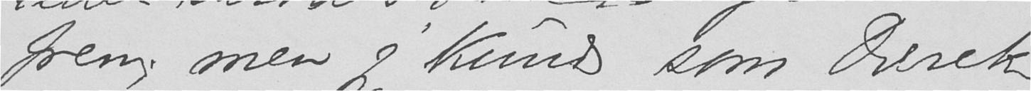frem, men jeg kunde som Direk-
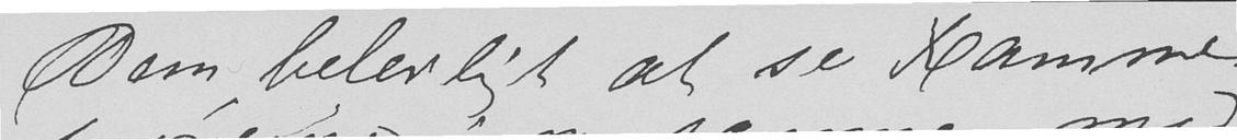Dem beleiligt at se Ramme-
